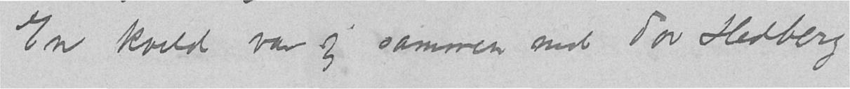En kveld var jeg sammen ned Tor Hedberg
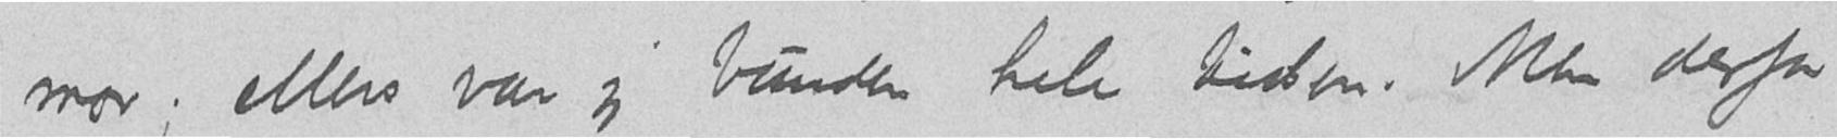mor; ellers var jeg bunden hele tiden. Men derfor
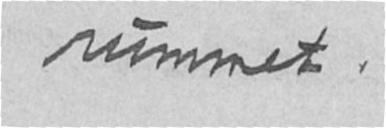rummet.
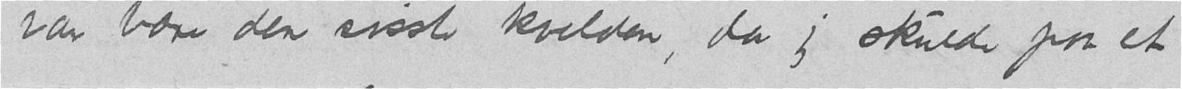var bare den sisste kvelden, da jeg skulde paa et
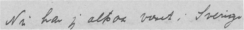Nu har jeg altsaa været i Sverige
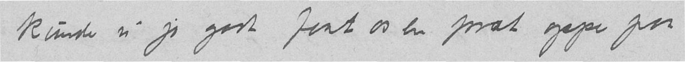kunde vi jo godt faat os en prat oppe paa
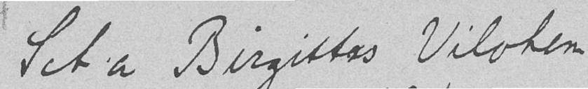Sct.a Birgittas Vilohem
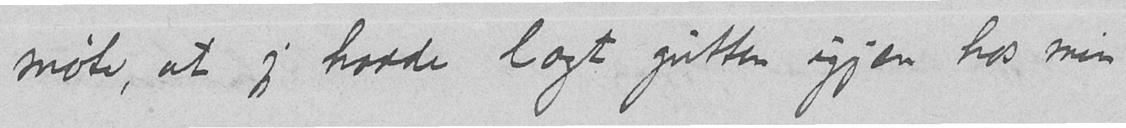møte, at jeg hadde lagt gutten igjen hos min
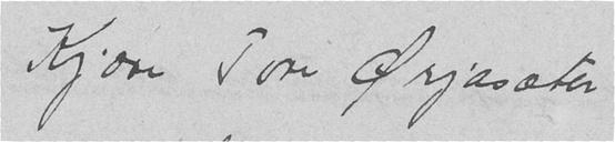Kjære Tore Ørjasæter
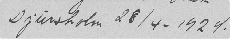Djursholm 28/4-1924.
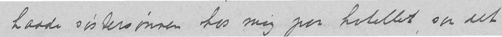hadde søstersønnen hos mig paa hotellet saa det
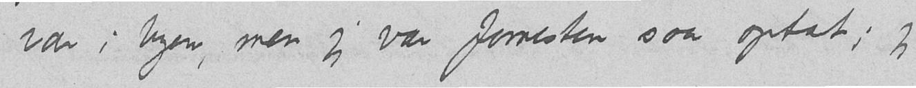var i byen, men jeg var forresten saa optat; jeg
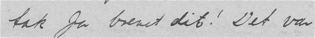tak for brevet dit! Det var
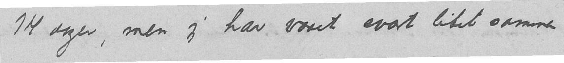14 dager, men jeg har været svært litet sammen
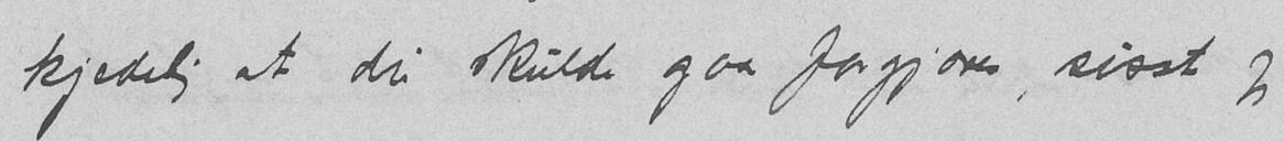kjedelig at du skulde gaa forgjæves, sisst jeg
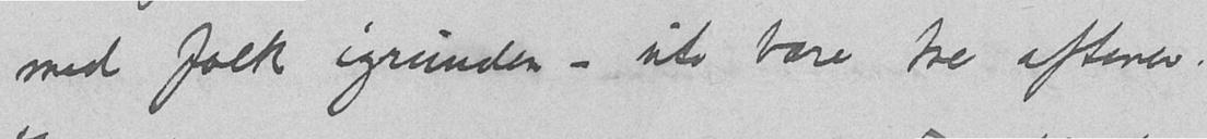med folk igrunden – ute bare tre aftener.
